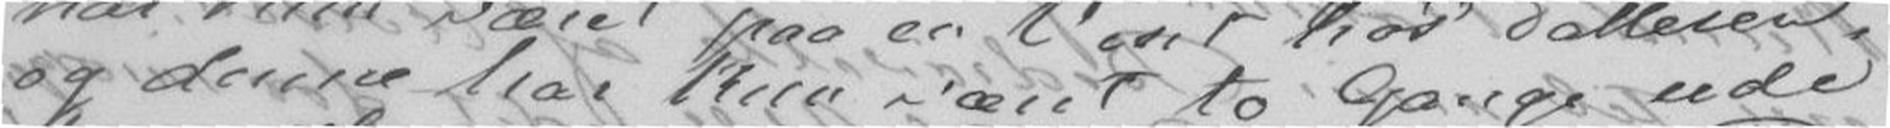og denne har kun været to Gange ude
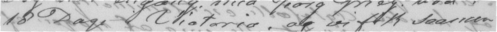18 Dage i Victoria, og vi fik sammen
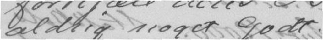aldrig noget godt. -
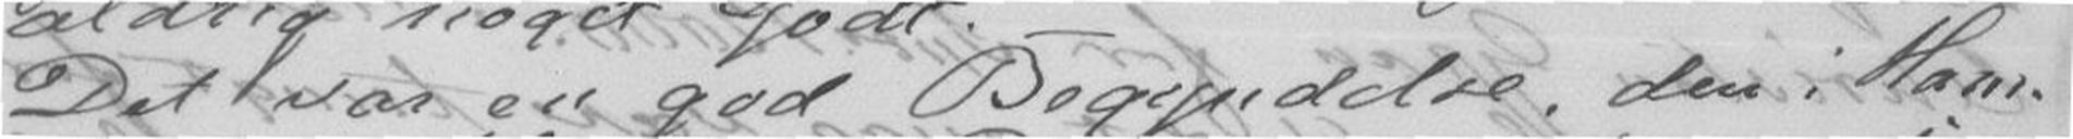Det var en god Begyndelse, den i Ham-
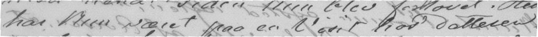har kun været paa en Visit hos Datteren,
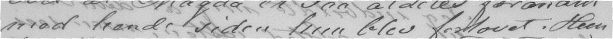mod hende siden hun blev forlovet. Hun
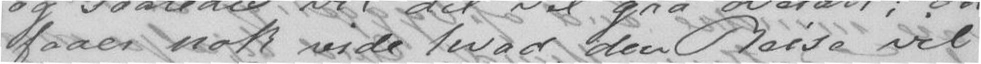faaer nok vide hvad den Reise vil
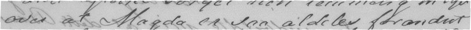over at Magda er saa aldeles forandret
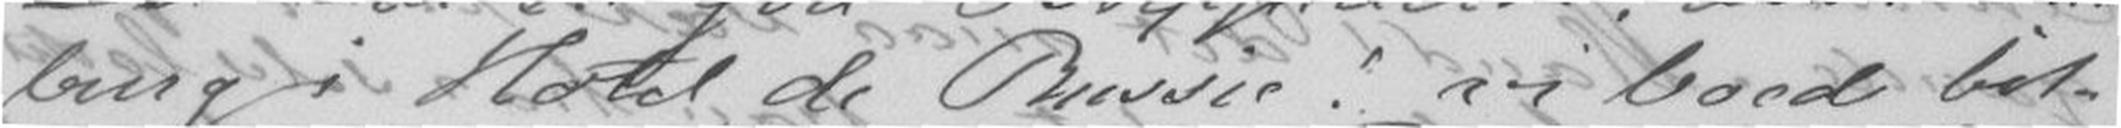burg i Hotel de Russie! vi boed bil-
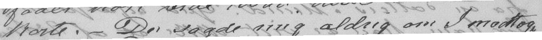koste. - Du sagde mig aldrig om I modtog
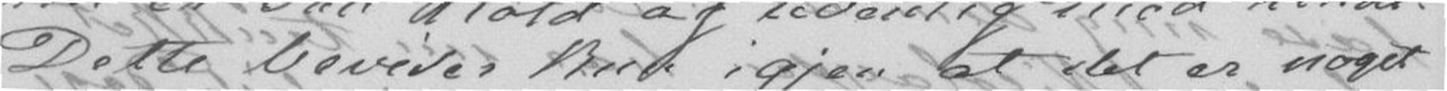Dette beviser kun igjen at det er noget
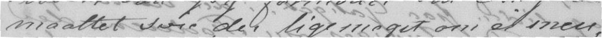maattet svie der ligemeget om ei mere,
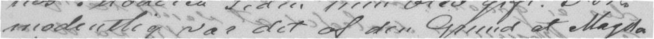modentlig var det af den Grund at Magda
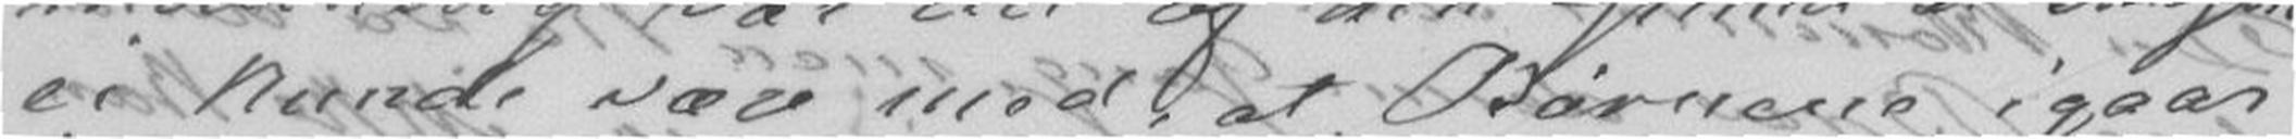ei kunde være med, at Børnene igaar
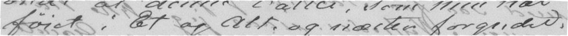føiet i Et og Alt, og næsten forgudet,
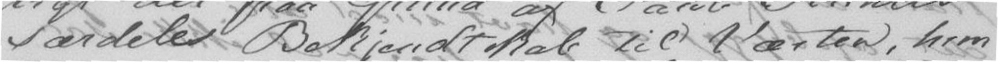særdeles Bekjentskab til Værten, hun
